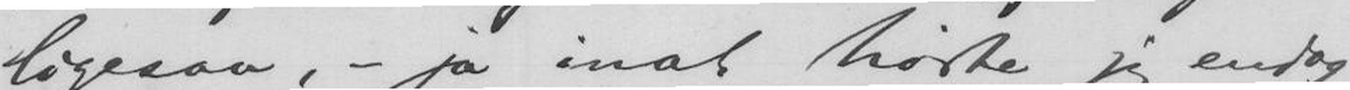ligesaa, - ja inat hørte jeg endog
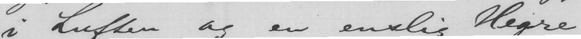i Luften og en enslig Hegre
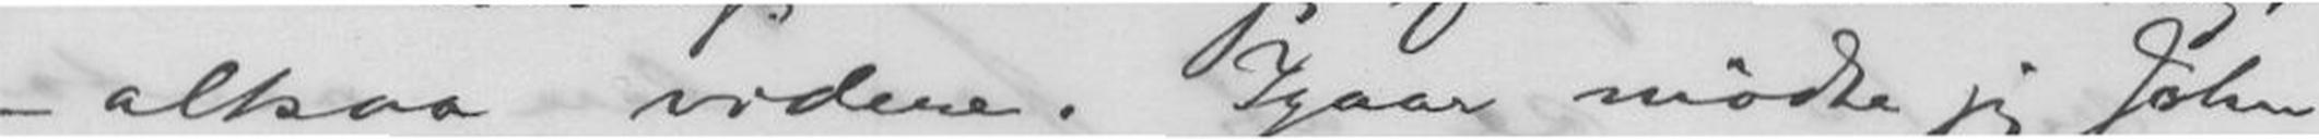- altsaa videre. Igaar mødte jeg John
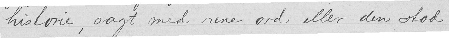historie, sagt med rene ord eller den stod
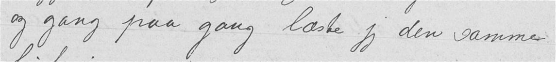og gang paa gang læste jeg den samme
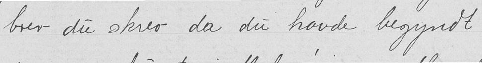brev du skrev da du havde begyndt
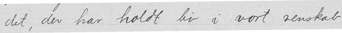det, der har holdt liv i vort venskab
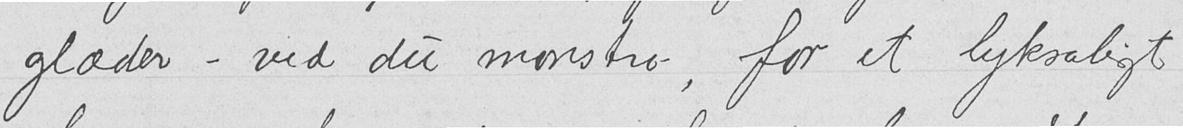glæder - ved du monstro, for et lyksaligt
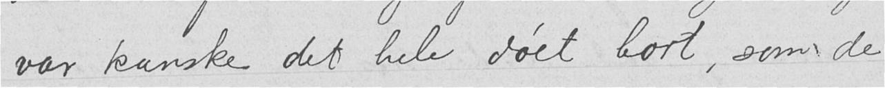var kanske det hele døet bort, som de
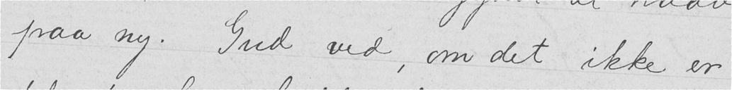paa ny. Gud ved, om det ikke er
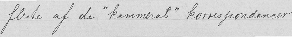fleste af de "kammerat" korrespondancer
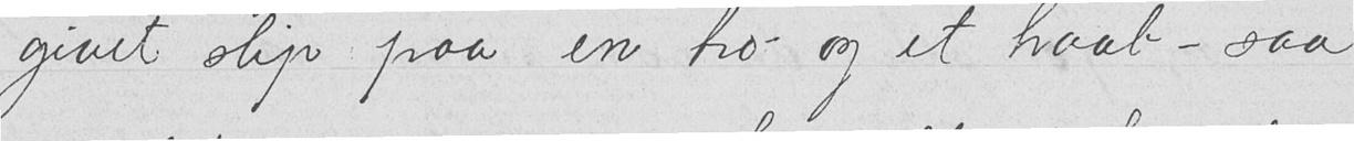givet slip paa en tro og et haab - saa
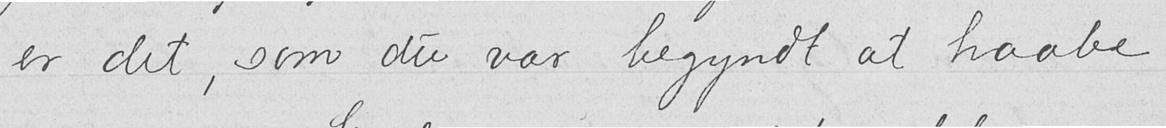er det, som du var begyndt at haabe
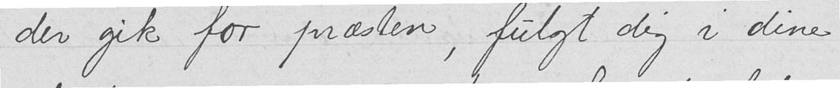der gik for præsten, fulgt dig i dine
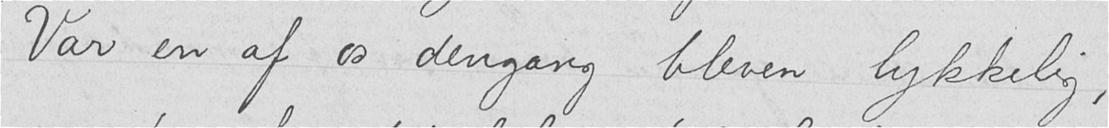Var en af os dengang bleven lykkelig,
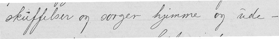skuffelser og sorger hjemme og ude -
